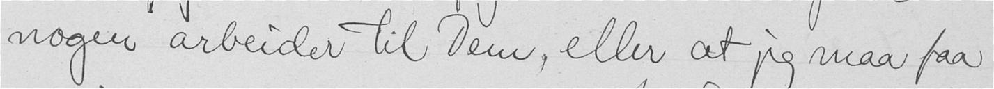nogen arbeider til Dem, eller at jeg maa faa
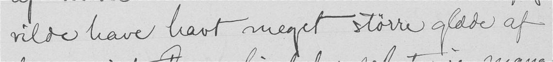vilde have havt meget større glæde af
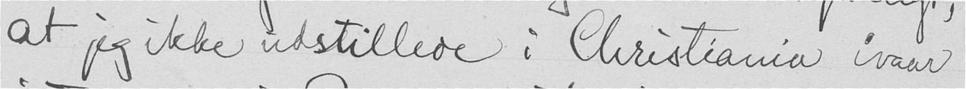at jeg ikke udstillede i Christiania ivaar
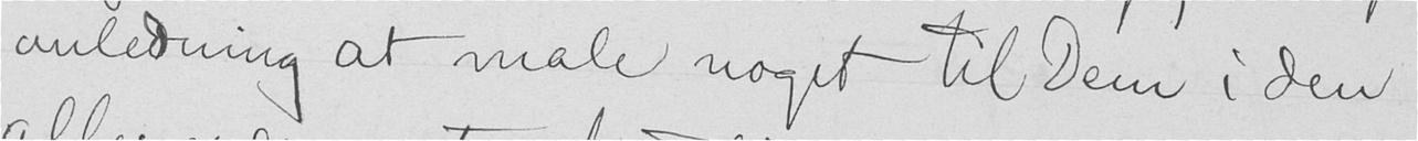anledning at male noget til Dem i den
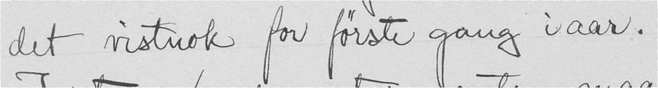det vistnok for første gang i aar.
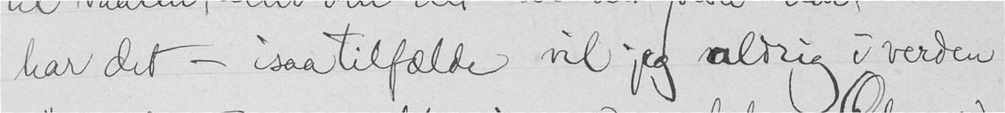har det - isaatilfælde vil jeg aldrig i verden
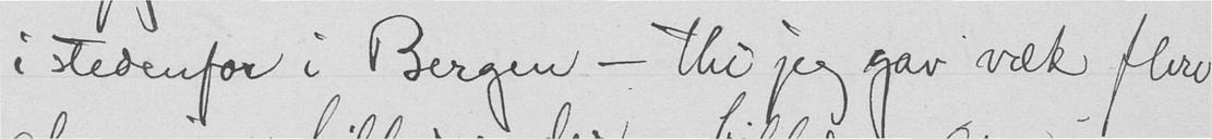i stedenfor i Bergen - thi jeg gav væk flere
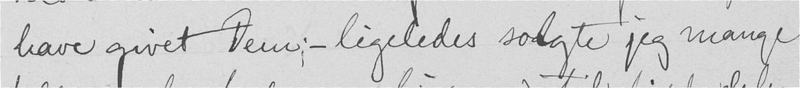have givet dem; - ligeledes solgte jeg mange
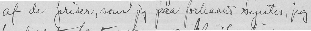af de priser, som jeg paa forhaand syntes, jeg
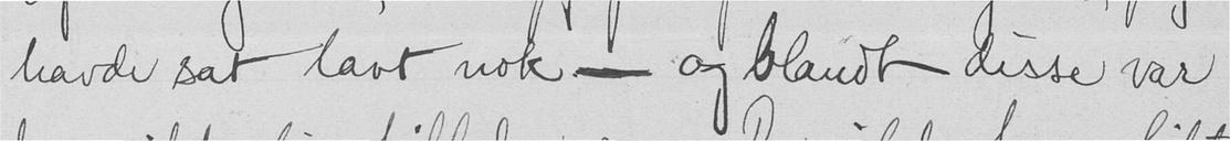havde sat lavt nok - og blandt disse var
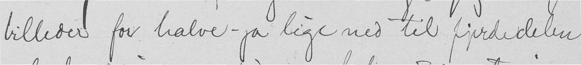billeder for halve - ja lige ned til fjerdedelen
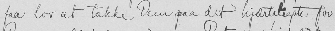faa lov at takke Dem paa det hiærteligste for
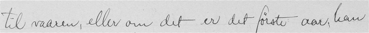til vaaren, eller om det er det første aar, han
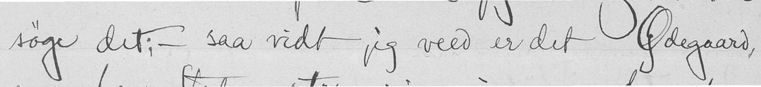søge det; - saa vidt jeg veed er det Ødegaard,
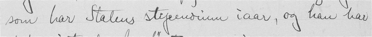som har Statens stipendium iaar, og han har
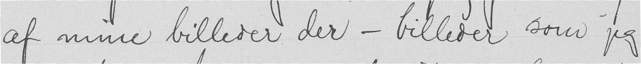af mine billeder der - billeder som jeg
